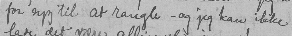for syg til at rangle - og jeg kan ikke
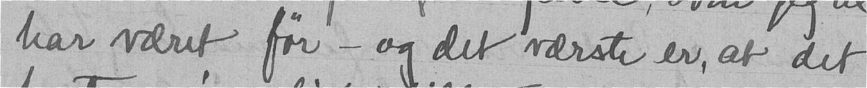har været før - og det værste er, at det
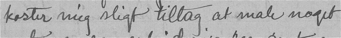korter mig sligt tiltag at male noget
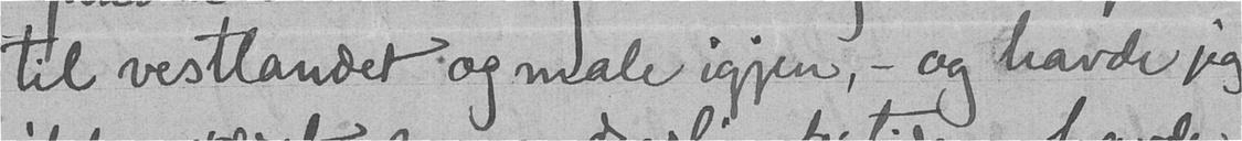til vestlandet og male igjen, - og havde jeg
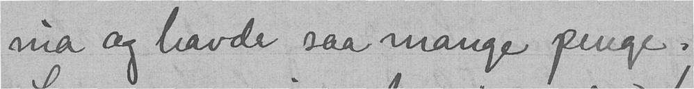nia og havde saa mange penge.
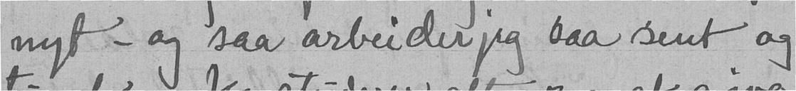nyt - og saa arbeider jeg saa sent og
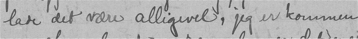lade det være alligevel, jeg er kommen
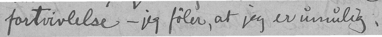fortvivlelse - jeg føler, at jeg er umulig.
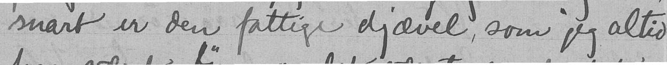snart er den fattige djævel, som jeg altid
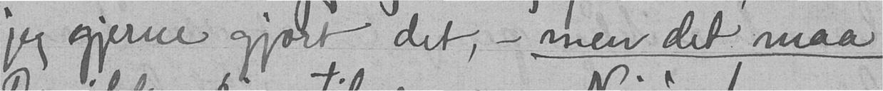jeg gjerne gjort det, - men det maa
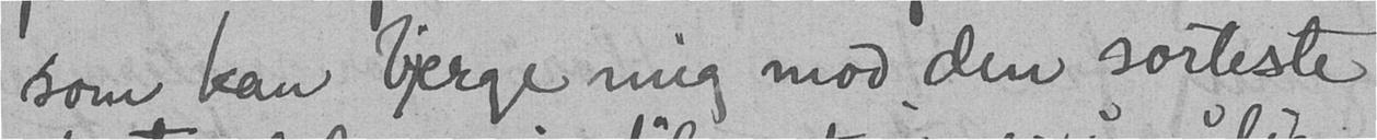som kan bjerge mig mod den sorteste
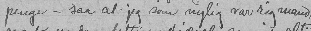penge - saa at jeg som nylig var rig mand,
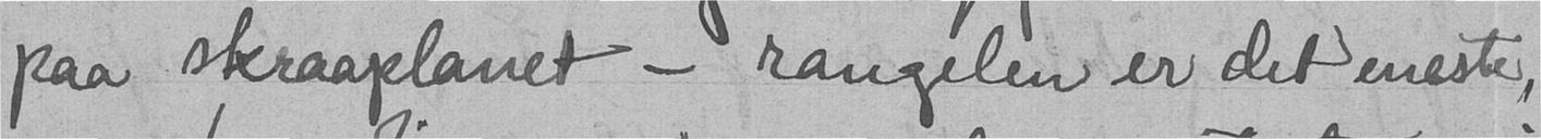paa skraaplanet - rangelen er det eneste,
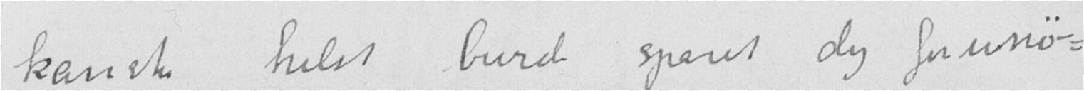kanske helst burde sparet dig for unø-
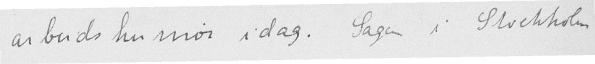arbeidshumør i dag. Sagen i Stockholm
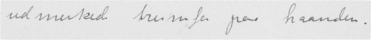udmerkede triumfer paa haanden.
